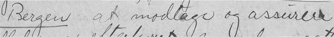Bergen at modtage og assurere
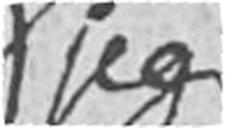jeg
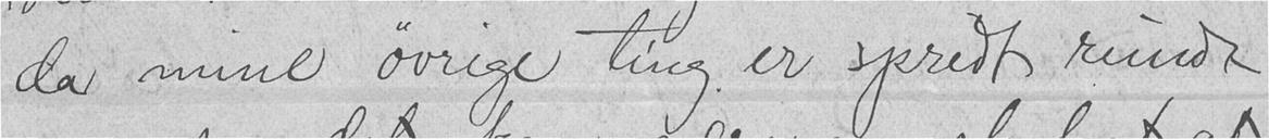da mine øvrige ting er spredt rundt
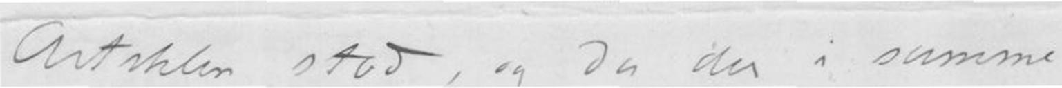Artiklen stod, og da der isamme
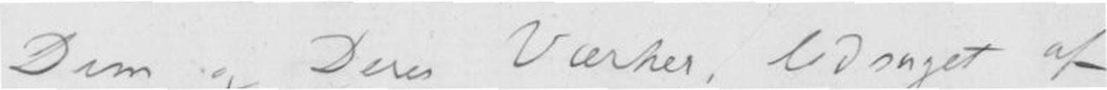Dem og Deres Værker, ledsaget af
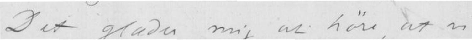Det glæder mig at høre, at vi
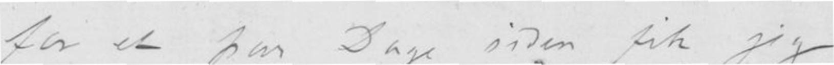for et par Dage siden fik jeg
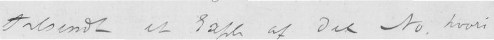tilksendt et Expl af det No. hvori
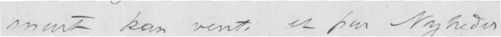snart kan vente et par Nyheder
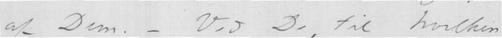af Dem. - Ved De, til hvilken
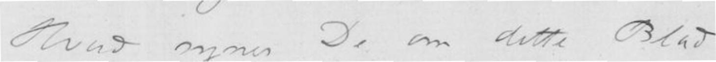Hvad synes De om dette Blad
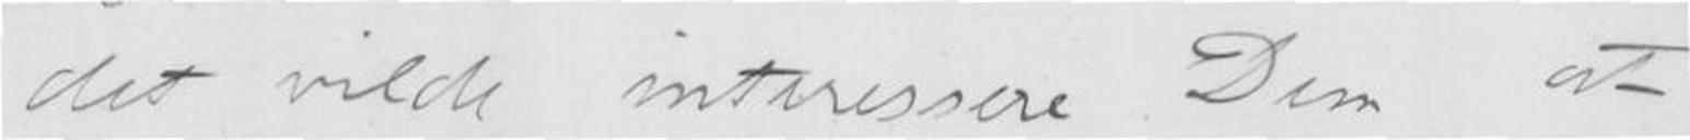det vilde interessere Dem at
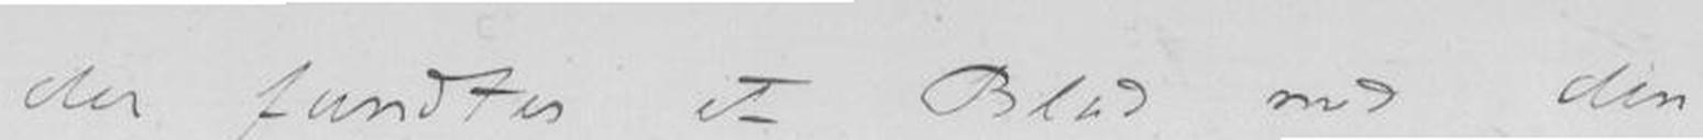der fantes et Blad med din
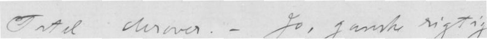Titel derover. - Jo, ganske rigtig, -
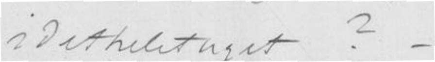idetheletaget? -
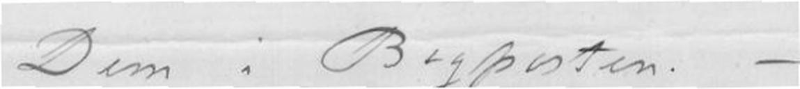Dem i Bogposten. -
